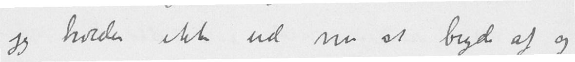jeg holder ikke ud nu at bryde af og
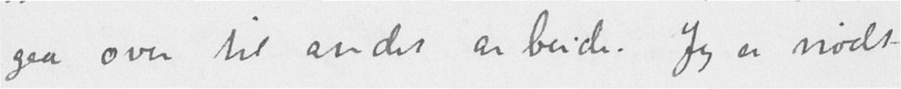gaa over til andet arbeide. Jeg er nødt
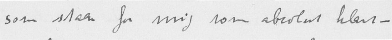som staar for mig som absolut klart -
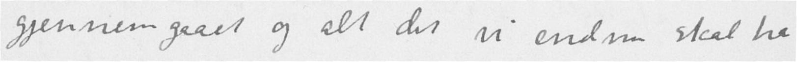gjennemgaaet og alt det vi endnu skal ha
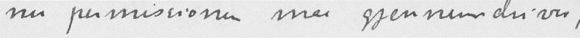nu permissionen maa gjennemdrives,
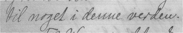til noget i denne verden.
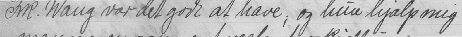Frk. Wang var det godt at have; og hun hjalp mig
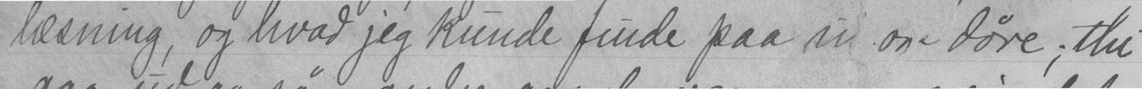læsning, og hvad jeg kunde finde paa in og døre; thi
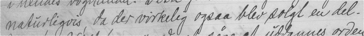naturligvis, da der virkelig ogsaa blev solgt en del.
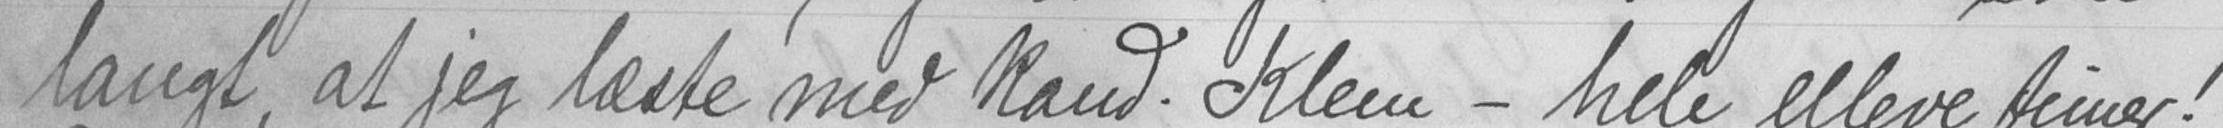langt, at jeg læste med kand. Klem - hele elleve timer!
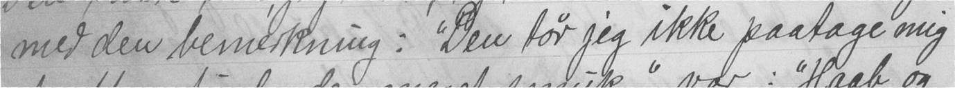med den bemerkning: "Den tør jeg ikke paatage mig
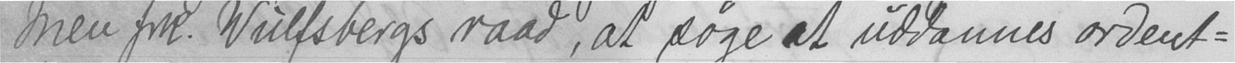Men frk. Wulfsbergs raad, at søge at uddannes ordent-
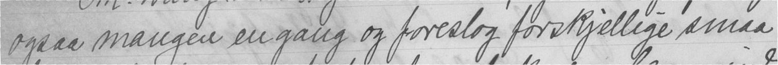ogsaa mangen en gang og foreslog forskjellige smaa
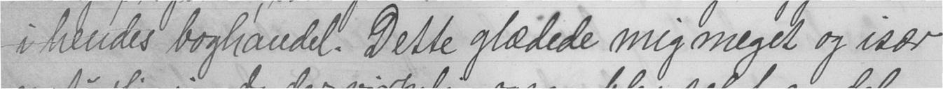i hendes boghandel. Dette glædede mig meget og især
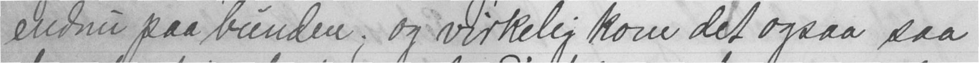endnu paa bunden; og virkelig kom det ogsaa saa
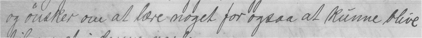og ønsker om at lære noget for ogsaa at kunne blive
